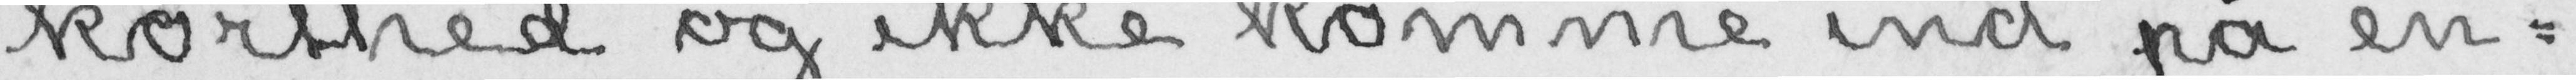korthed og ikke komme ind på en-
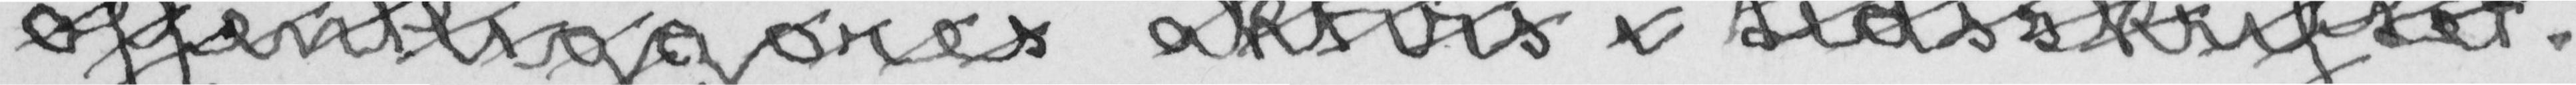offentliggøres aktvis i tidsskriftet.
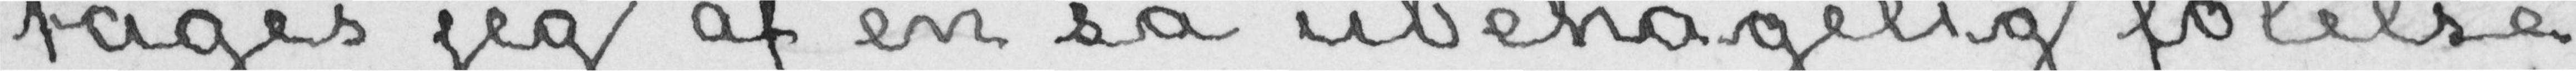tages jeg af en så ubehagelig følelse
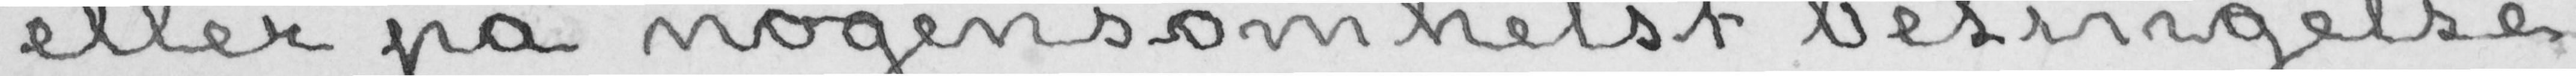eller på nogensomhelst betingelse
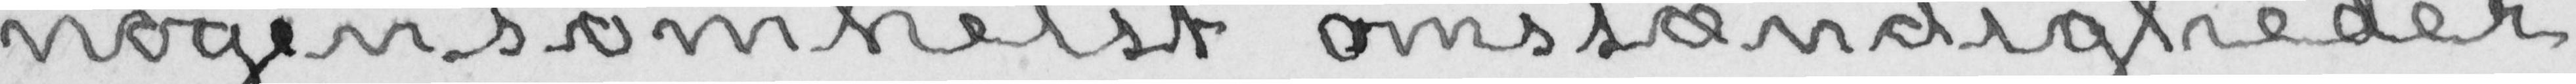nogensomhelst omstændigheder
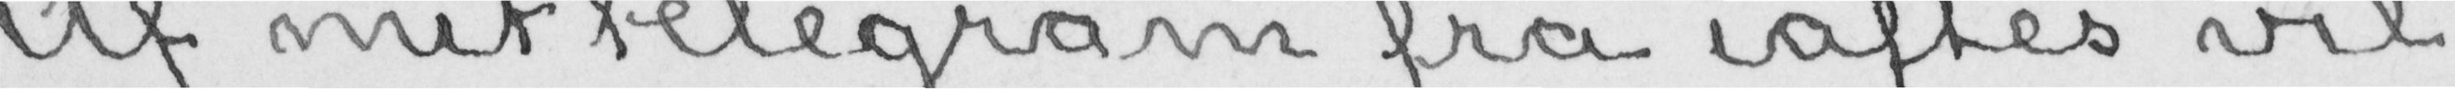Af mit telegram fra iaftes vil
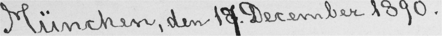München, den 17. December 1890.
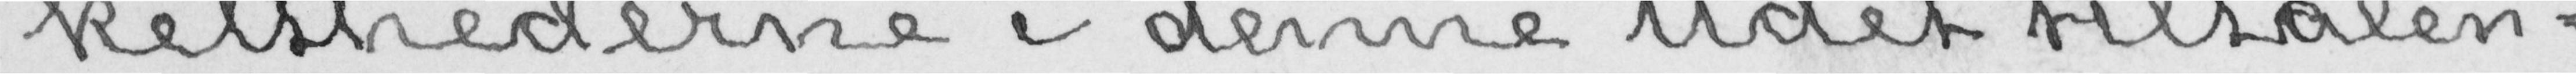kelthederne i denne lidet tiltalen-
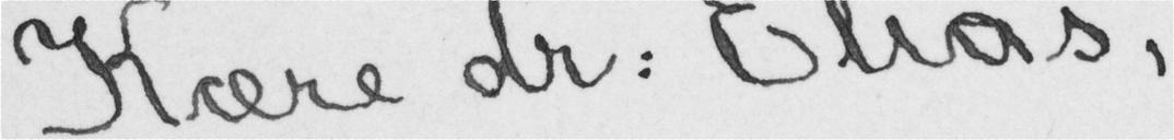Kære dr: Elias,
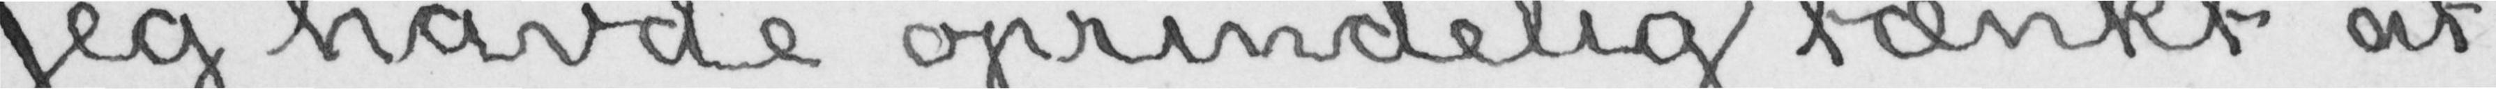Jeg havde oprindelig tænkt at
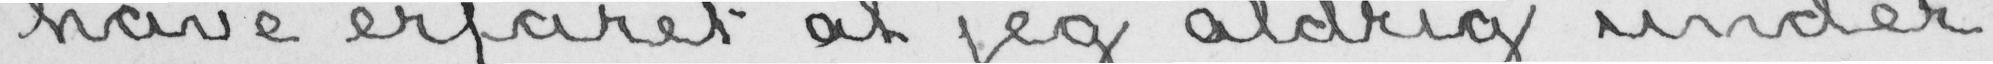have erfaret at jeg aldrig under
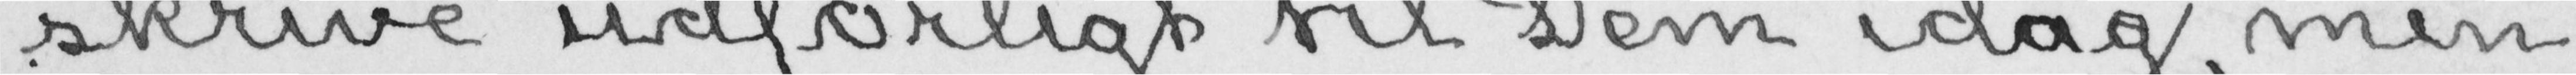skrive udførligt til Dem idag, men
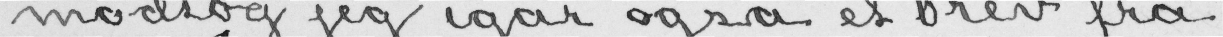modtog jeg igår også et brev fra
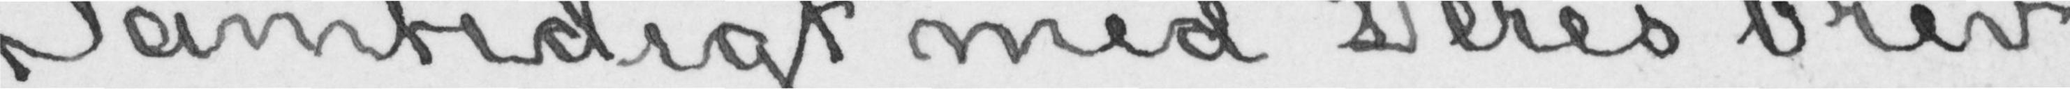Samtidigt med Deres brev
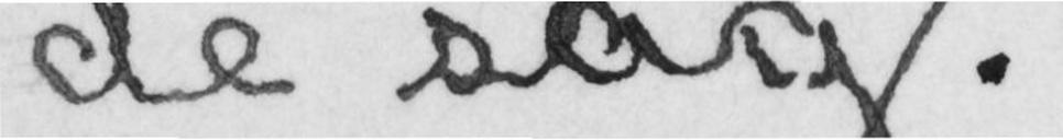de sag.
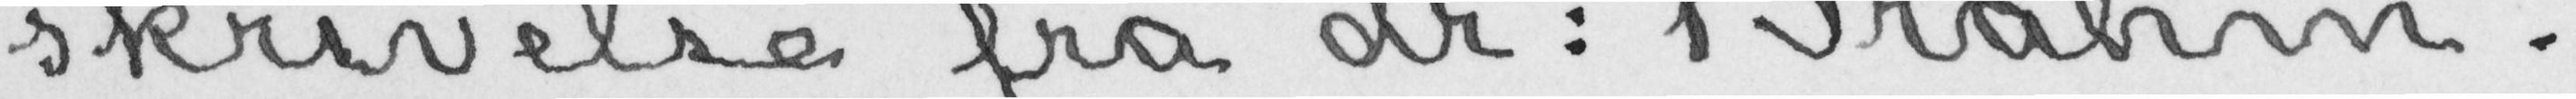skrivelse fra dr: Brahm.
